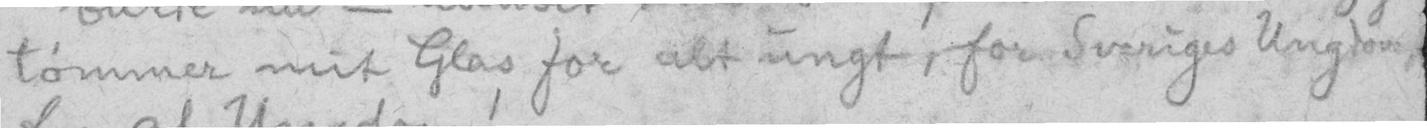tømmer mit Glas for alt ungt, for Sveriges Ungdom,
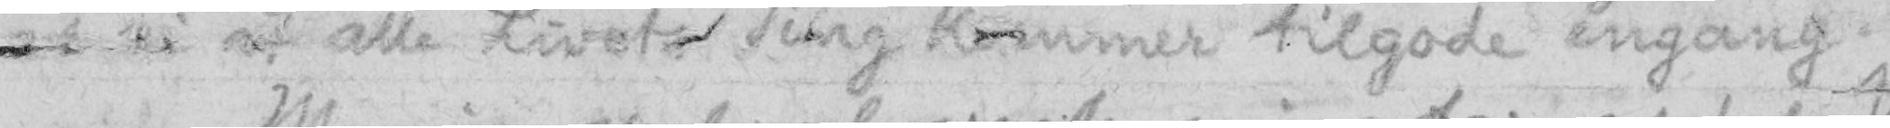at si at alle Livets Ting kommer tilgode engang.
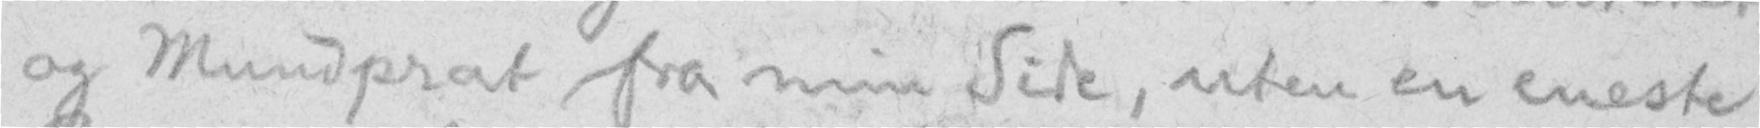og Mundprat fra min Side, uten en eneste
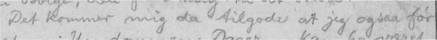Det kommer mig da tilgode at jeg ogsaa før
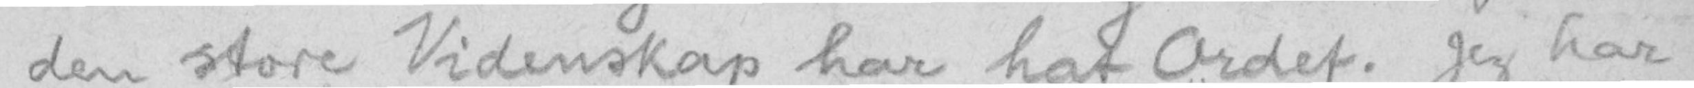den store Videnskap har hat Ordet. Jeg har
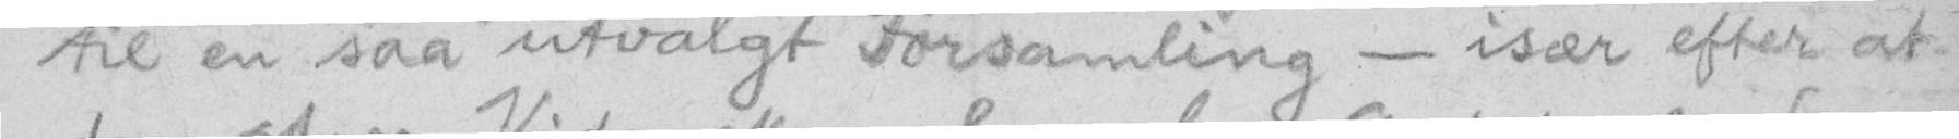til en saa utvalgt Forsamling - især efter at
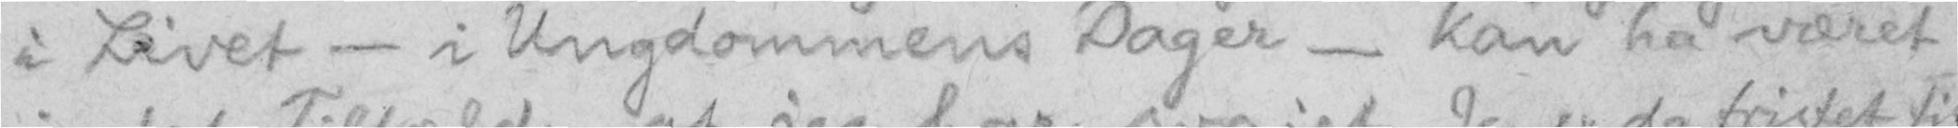i Livet - i Ungdommens Dager - kan ha været
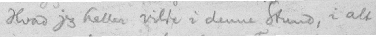Hvad jeg heller vilde i denne Stund, i alt
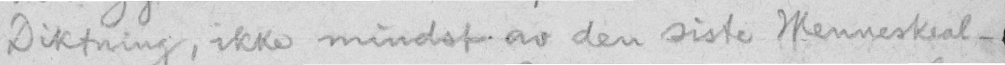Diktning, ikke mindst av den siste Menneskeal-
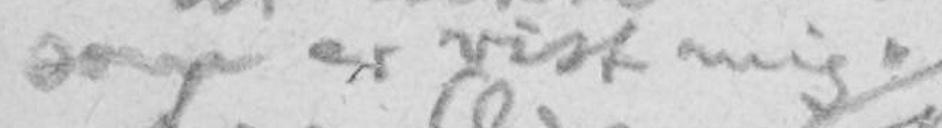som er vist mig,
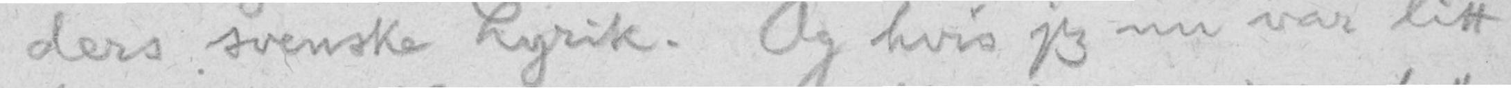ders svenske Lyrik. Og hvis jeg nu var litt
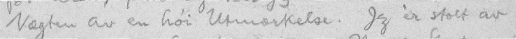Vægten av en høi Utmærkelse. Jeg er stolt av
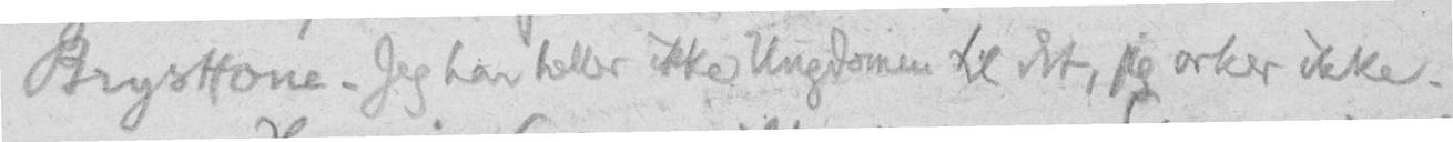Brysttone. Jeg har heller ikke Ungdomen til det, jeg orker ikke.
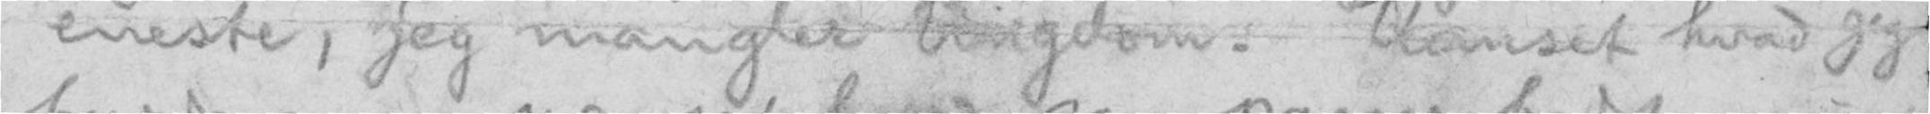eneste, jeg mangler Ungdom. Uanset hvad jeg
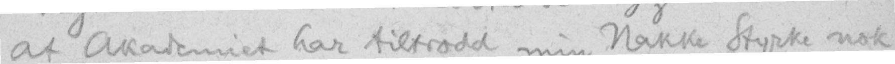at Akademiet har tiltrodd min Nakke Styrke nok
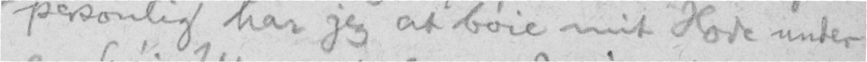personlig har jeg at bøie mit Hode under
